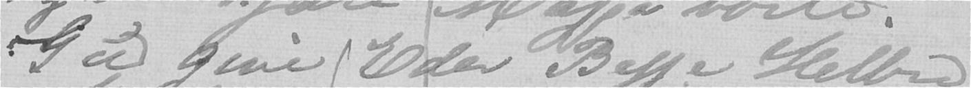Gud give Eder Begge Helbred
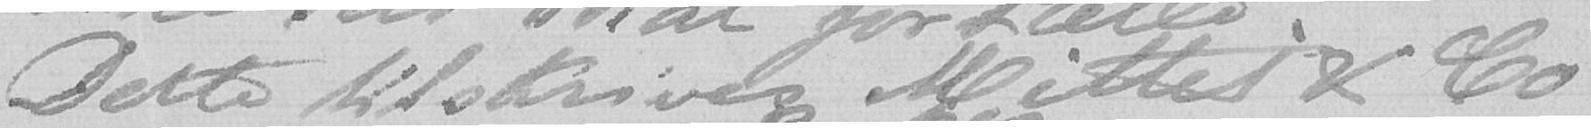Dette tilskrives Mittet & Co
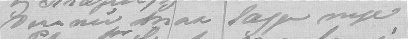Dere nu maa lægge nye
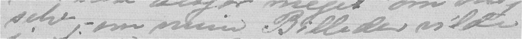selv, - om mine Billeder vilde
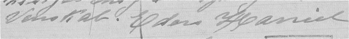Venskab. Eders Harriet
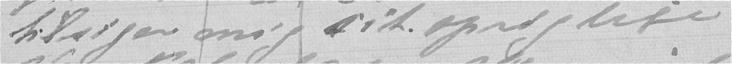tilsiger mig sit oprigtige
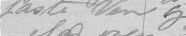faste Ven, og
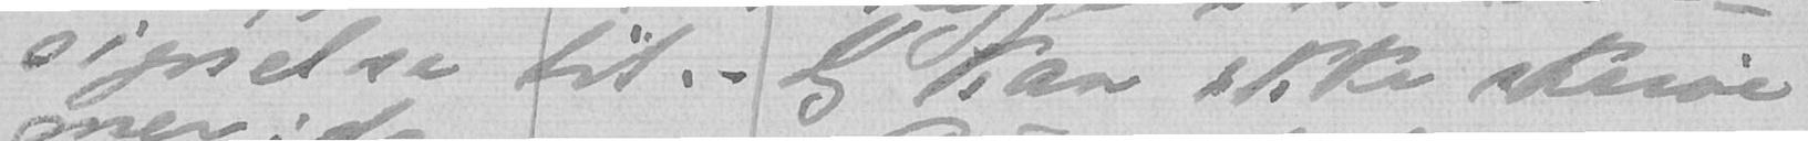signelse til. Jeg kan ikke skrive
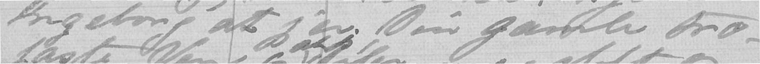lngeborg at jeg er Din gamle tro-
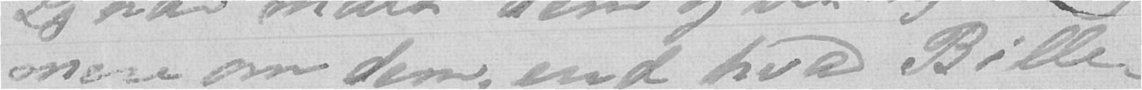mere om dem, end hvad Bille-
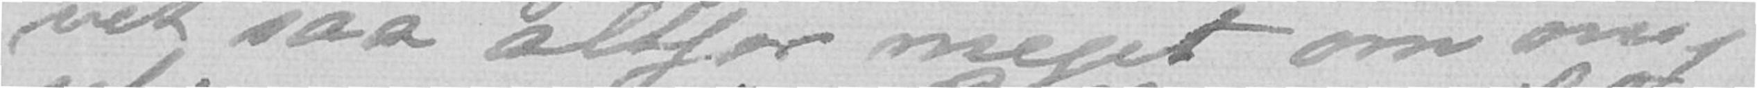vet saa altfor meget om mig
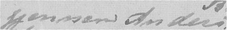gjennem Anders
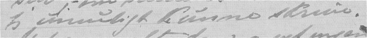jeg umuligt kunne skrive.
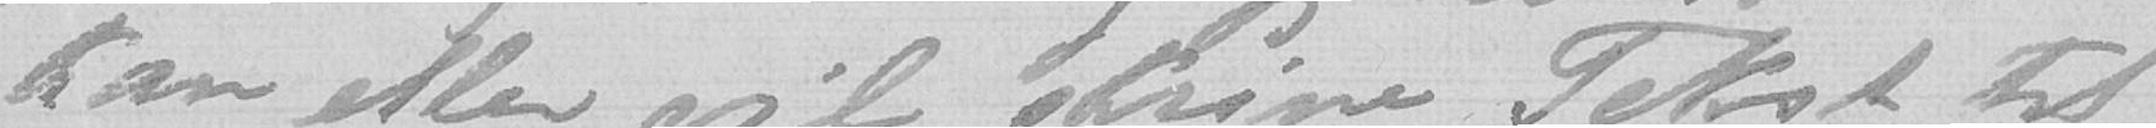kan eller vil skrive Tekst til
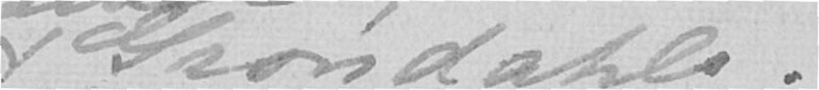Grøndahls.
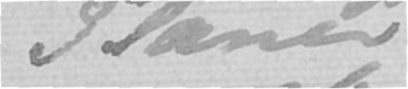Planer
